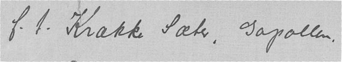f.t. Krække Sæter, Gopollen.
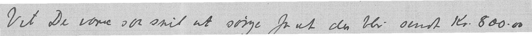Vil De være saa snil at sørge for at der blir sendt kr. 800.00
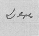Deres
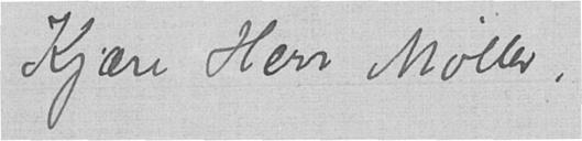Kjære Herr Møller,
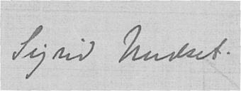Sigrid Undset.
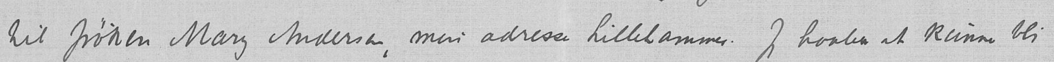til frøken Mary Andersen, min adresse Lillehammer. Jeg haaber at kunne bli
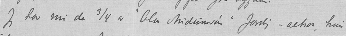Jeg har nu de 3/4 av "Olav Audunssøn" færdig – altsaa, hvis
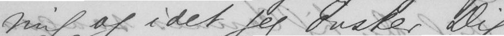mig og idet jeg ønsker Dig
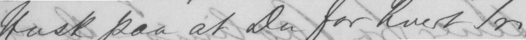Husk paa at Du for hvert Tri-
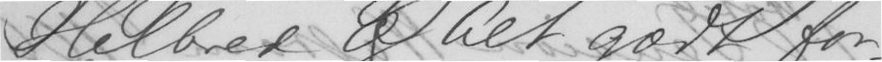Helbred og Alt godt for-
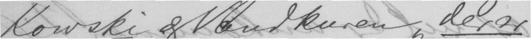Kowski og Vandkuren, der er
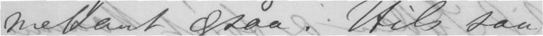mekant ogsaa. Hils saa
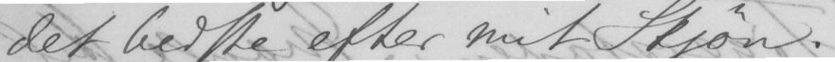det bedste efter mit Skjøn.
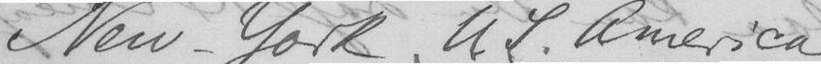New-York U S. America
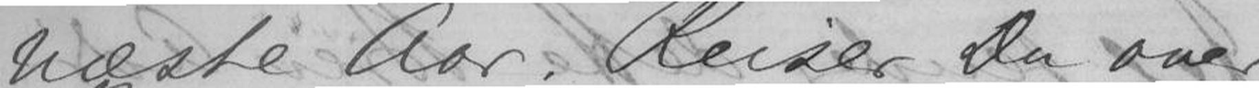næste Aar. Reiser Du over
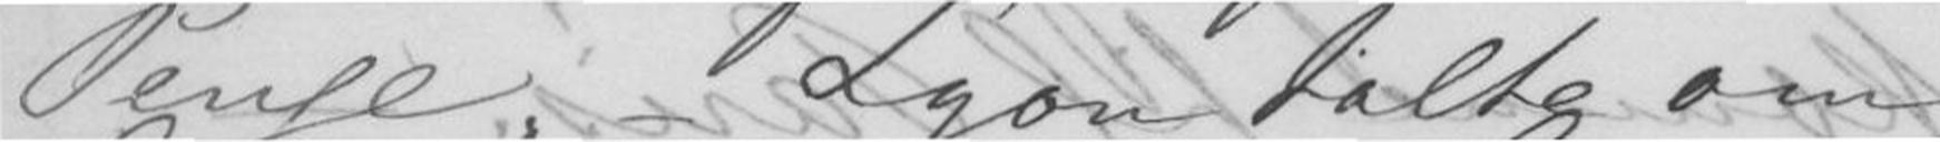Penge. - Lyon talte om
